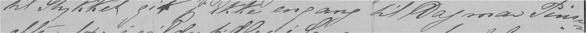til Stykket gik jeg ikke engang til Dagmar Pinse-
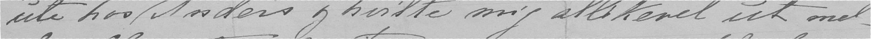ute hos Anders og hvilte mig allikevel ut mel-
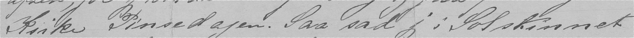Kirke Pinsedagen. Saa sad jeg i Solskinnet
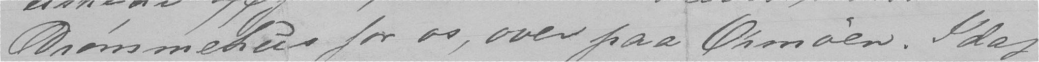Drømmehus for os over paa Ormøen. Idag
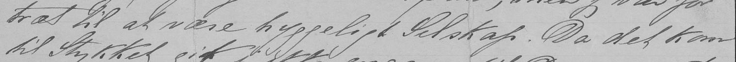træt til at være hyggeligt Selskap. Da det kom
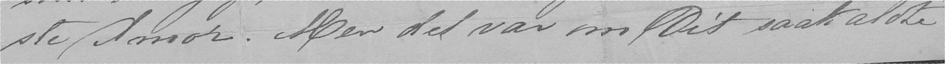ste Amor. Men det var om Dit saakaldte
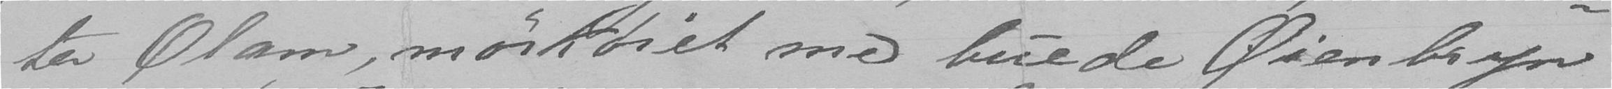ter Olam, mørkøiet med buede Øienbryn
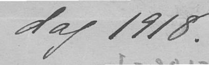dag 1918.
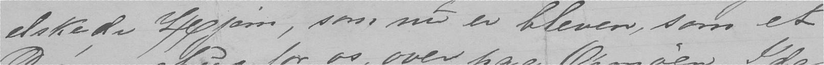elskede Hjem, som nu er bleven, som et
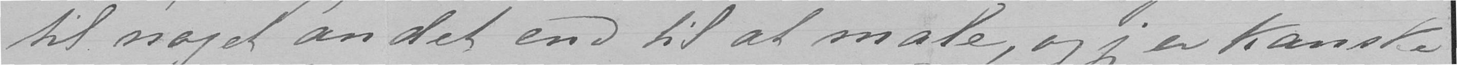til noget andet end til at male, og jeg er kanske
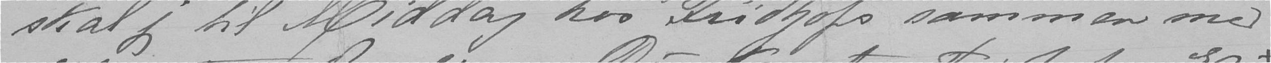skal jeg til Middag hos Fridtjofs sammen med
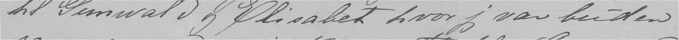til Gunwald og Elisabet hvor jeg var buden
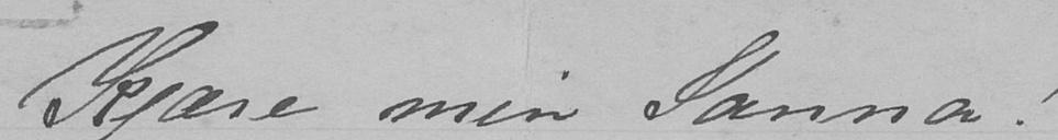Kjære min Janna!
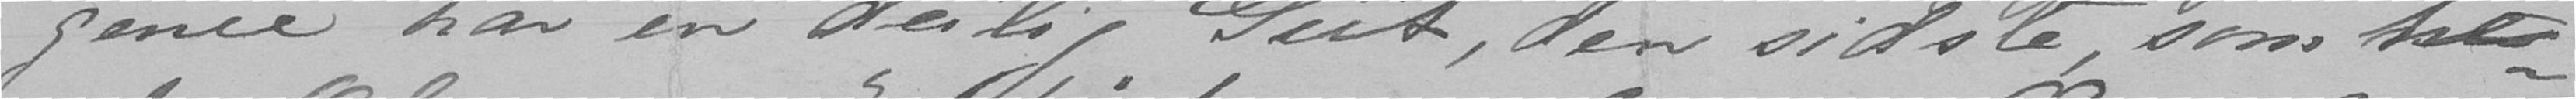génie har en deilig Gut, den sidste, som he-
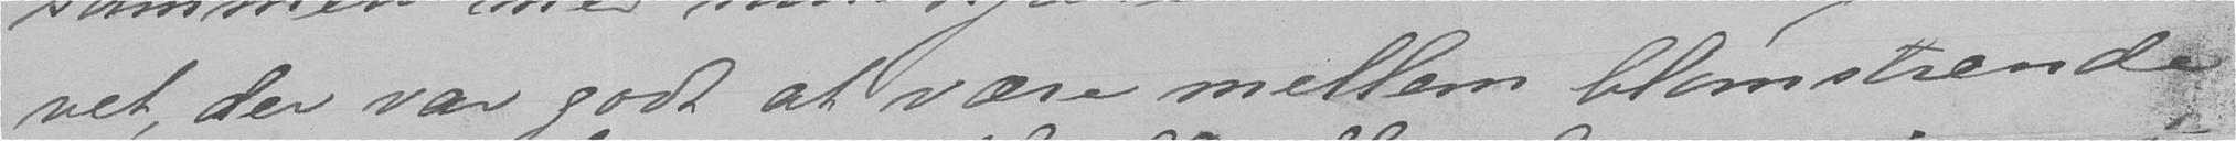vet, der var godt at være mellem blomstrende
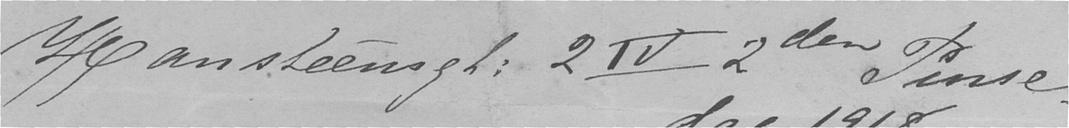Hansteensgt. 2 IV 2den Pinse-
